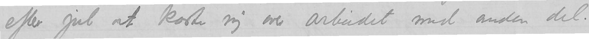efter jul at kaste mig over arbeidet med anden del.
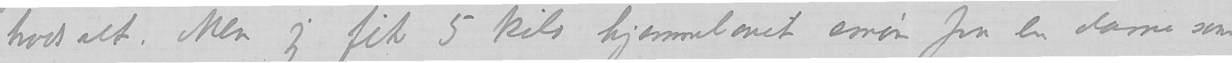trods alt.  Men jeg fik 5 kilo hjemmelavet smør fra en dame som
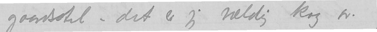gaardsstel – det er jeg vældig kry av. Jeg glæder mig vildt til
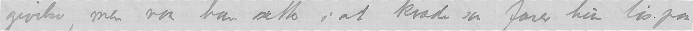givelse, men naar han sætter i at kvæde saa farer hun løs paa
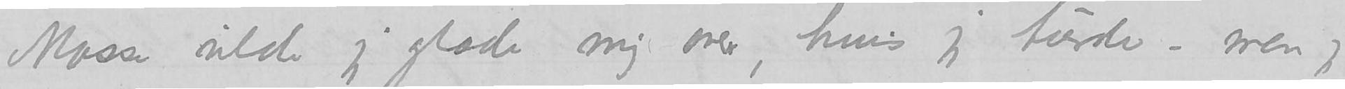Mosse vilde jeg glæde mig over, hvis jeg turde –, men jeg
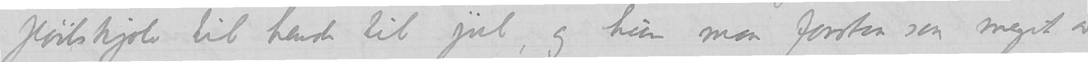fløiskjole til hende til jul, og hun maa forstaa saa meget av teksterne at
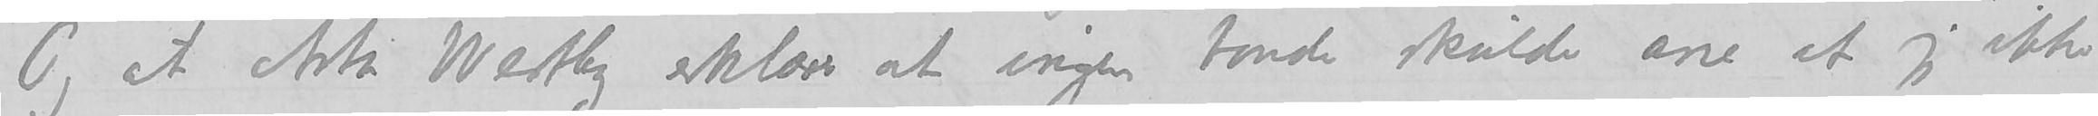Og at Asta Westby erklærer at ingen bonde skulde ane at jeg ikke
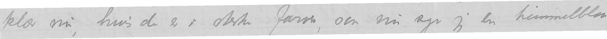klær nu, hvis de er i sterke farver, saa nu syr jeg en himmelblaa
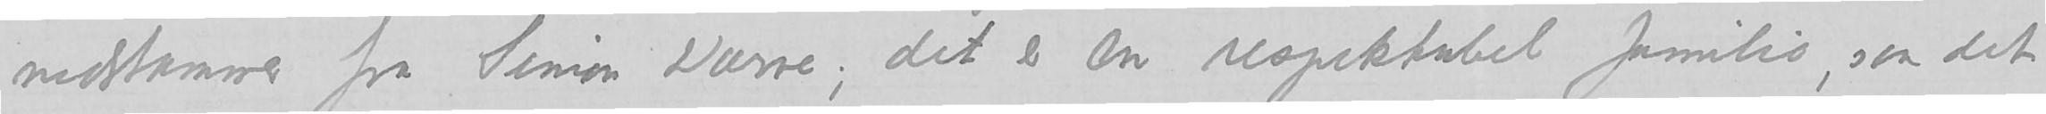nedstammer fra Simon Darre; det er en respektabel familie, saa det
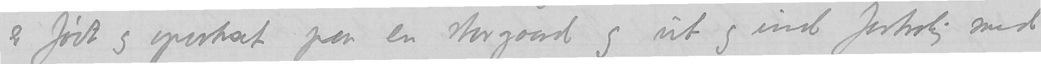er født og opvokset paa en storgaard og ut og ind fortrolig med
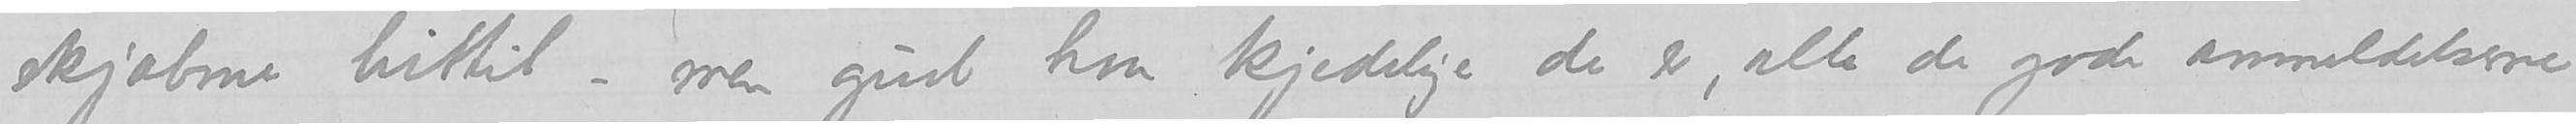skjæbne hittil – men gud hvor kjedelige de er, alle de gode anmeldelserne
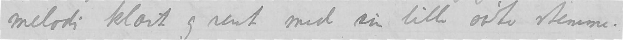melodi klart og rent med sin lille søte stemme.
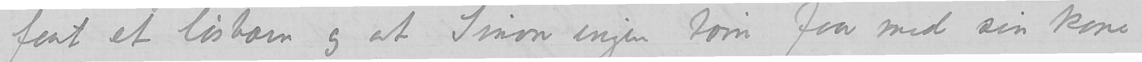faat et løsbarn og at Simon ingen barn faar med sin kone.
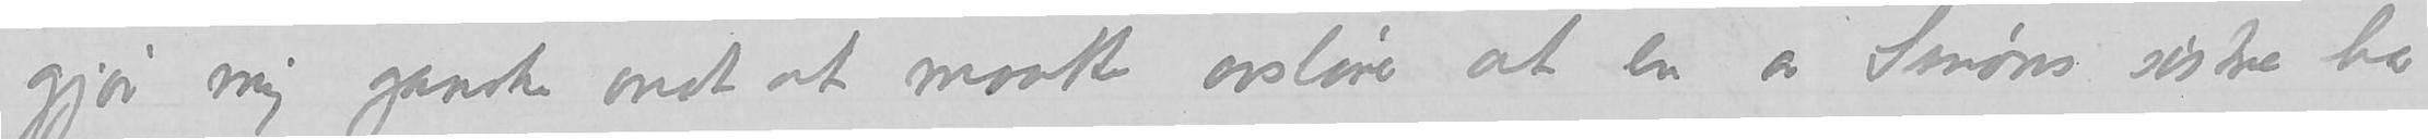gjør mig ganske ondt at maatte avsløre at en av Simons søstre har
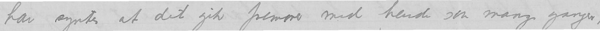har syntes at det gik fremover med hende saa mange ganger,
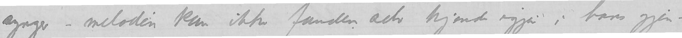synger – melodien kan ikke fanden selv kjende igjen i hans gjen-
